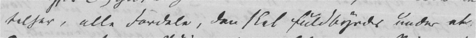telser, alle Fordele, den skal fuldbyrdes under et
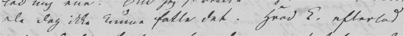De dog ikke kunne fatte det. Hvad C. efterlod
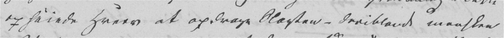ophøiede Hverv at opdrage Slægten – deriblandt maaskee
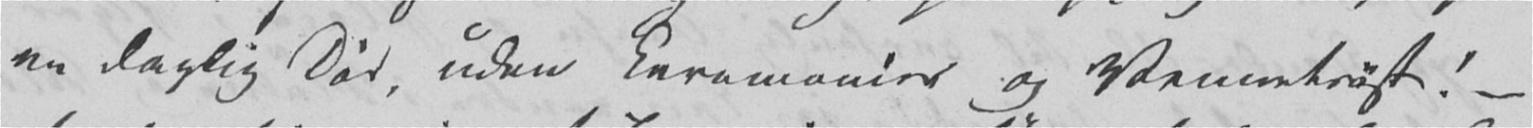en daglig Død, uden Ceremonier og Vennetrøst! –
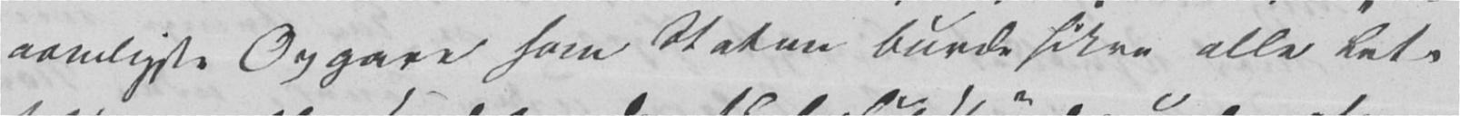aandigste Opgave som Staten burde sikre alle Let-
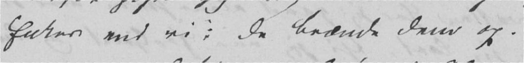Enker end vi: de brænde dem op.
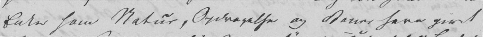Enker som Natur, Opdragelse og Vaner have givet
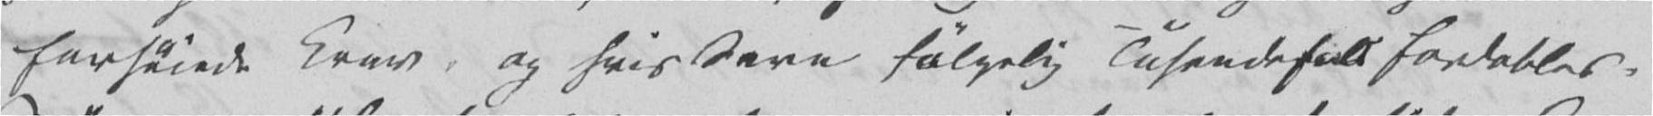forhøiede Krav, og hvis Savn følgelig Tusendefold fordobles.
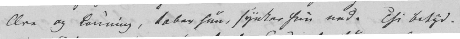Ære og Lønning, taber hun, synker hun ned. Thi betyd-
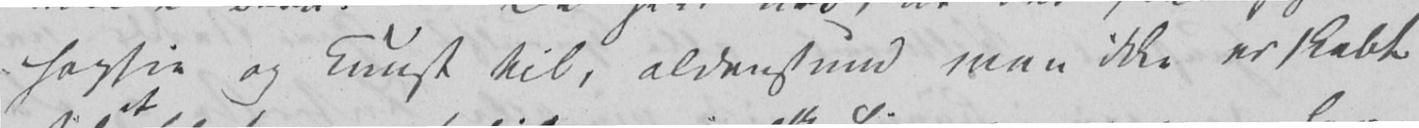sophie og Kunst til, aldenstund man ikke er skabt
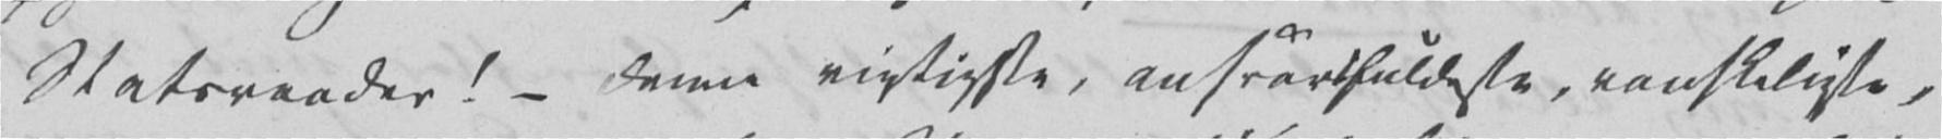Statsraader! – denne vigtigste, ansvarsfuldeste, vanskeligste,
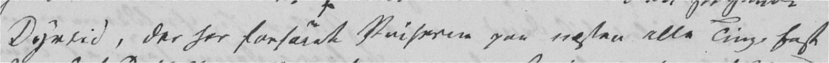Dyrtid, der har forhøiet Priserne paa næsten alle Ting, fast
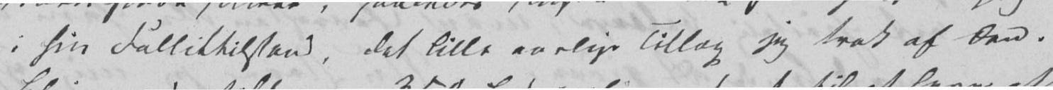i sin Fallittilstand, det lille aarlige Tillæg jeg trak af den.
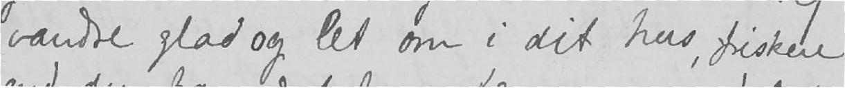vandre glad og let om i dit hus, friskere
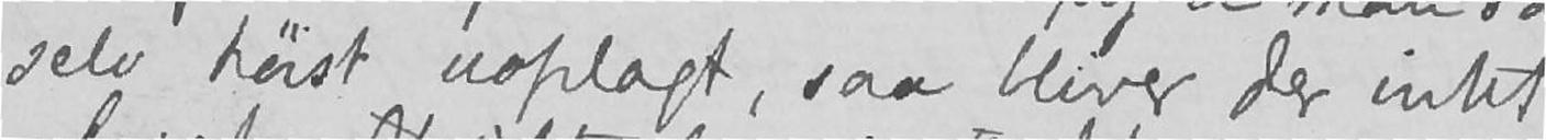selv høist uoplagt, saa bliver der intet
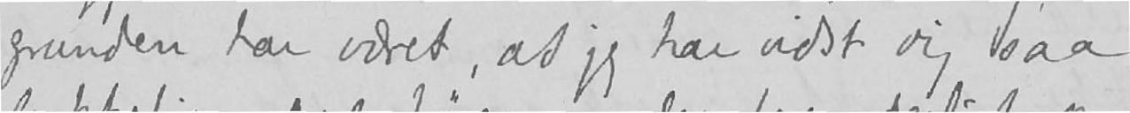grunden har været, at jeg har vidst dig saa
